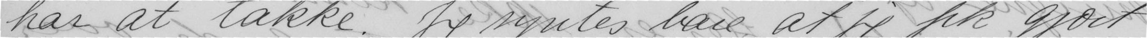har at takke. Jeg syntes bare at jeg fik gjort
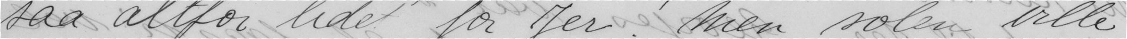saa altfor lidet for Jer! Men solen ville
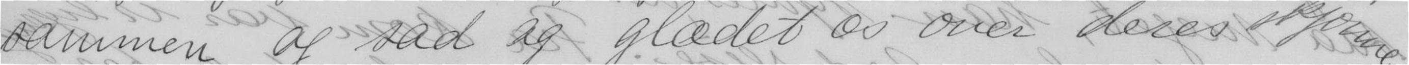sammen og sad og glædet os over deres skjønne
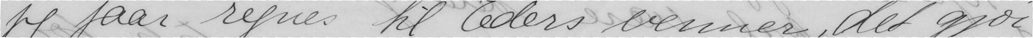jeg faar regnes til Eders venner, det gjør
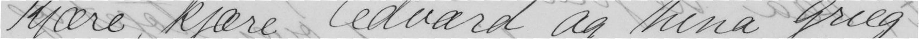Kjære, kjære Edvard og Nina Grieg!
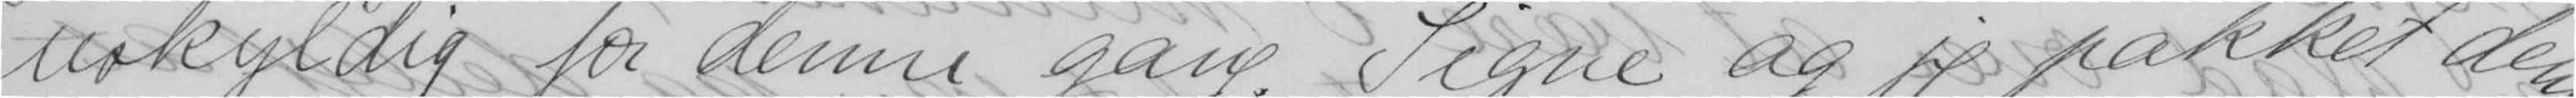uskyldig for denne gang. Signe og jeg pakket dem
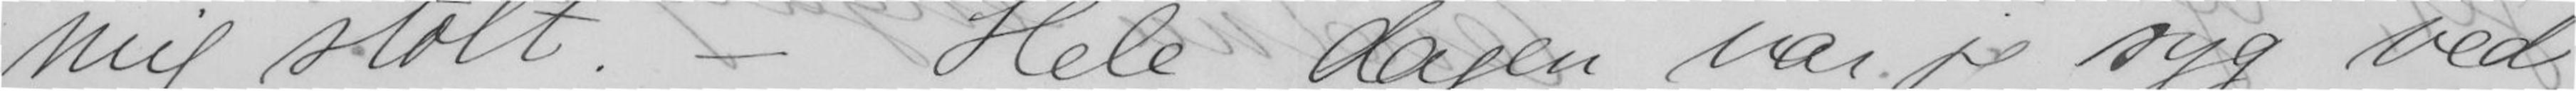mig stolt. - Hele dagen var jeg syg ved
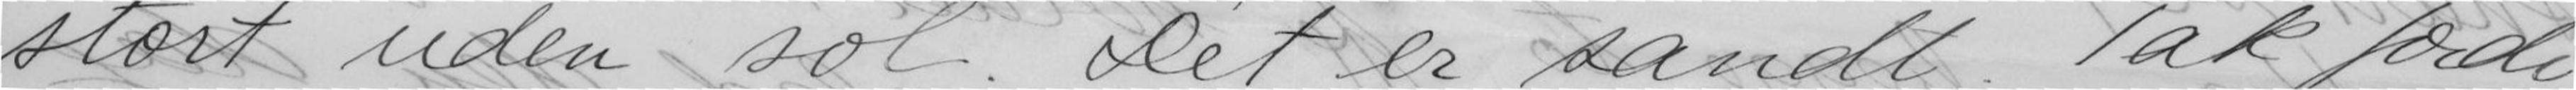stort uden sol. Det er sandt. Tak fordi
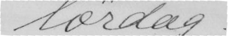lørdag
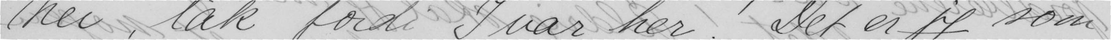Nei, tak fordi I var her! Det er jeg som
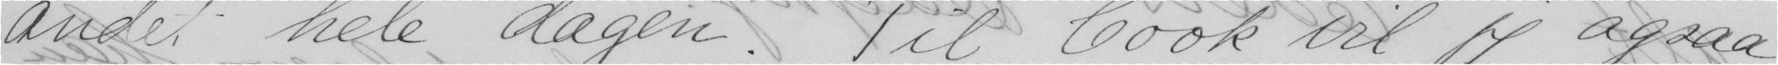andet hele dagen. Til Cook vil jeg ogsaa
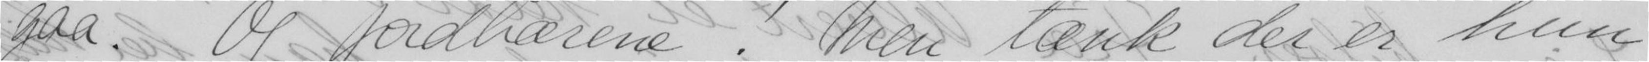gaa. Og jordbærene! Men tænk der er hun
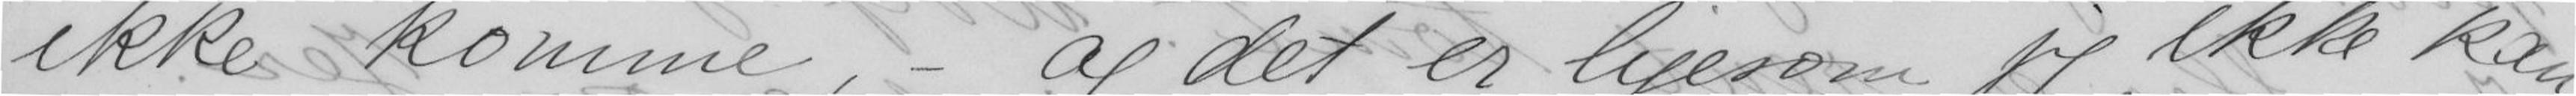ikke komme, - og det er ligesom jeg ikke kan
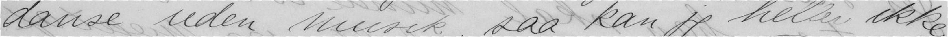danse uden musik, saa kan jeg heller ikke
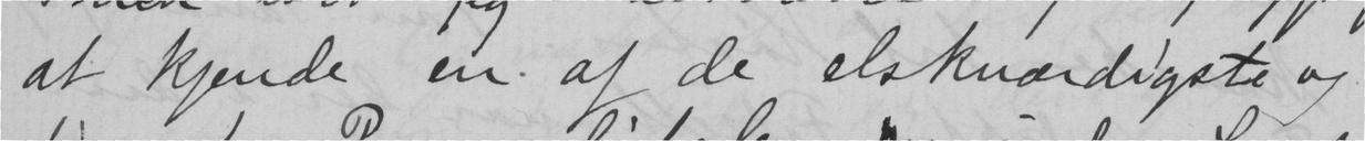at kjende en af de elskværdigste og
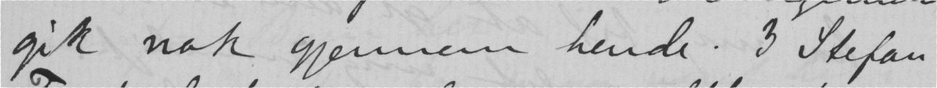gik nok gjennem hende. I Stefan
